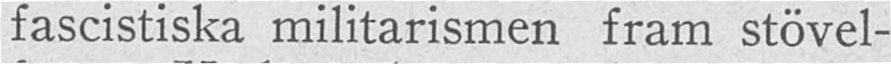fascistiska militarismen fram stövel-
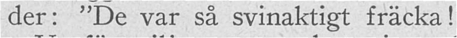der: "De var så svinaktigt fräcka!"
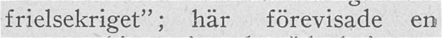frielsekriget" här forevisade en
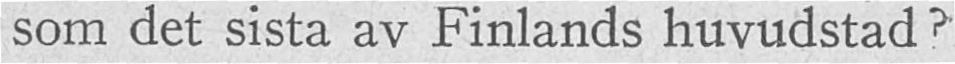som det sista av Finlands huvudstad?
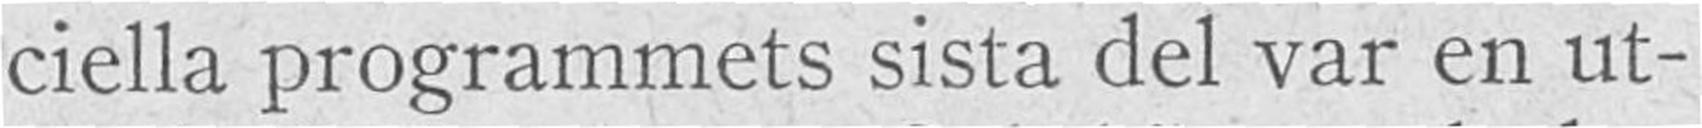ciella programmets sista del var en ut-
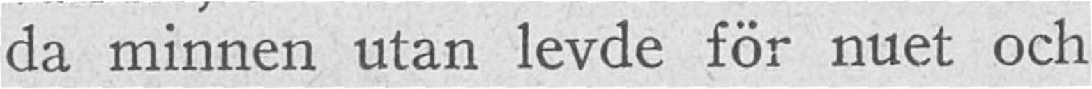da minnen utan levde för nuet och
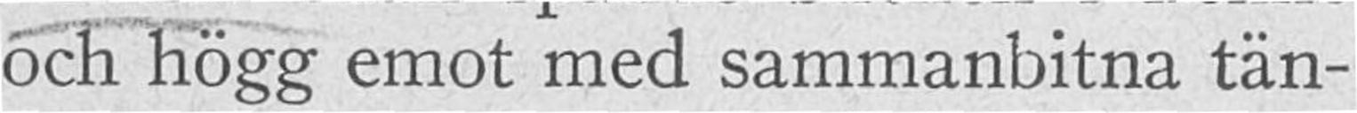och högg emot med sammanbitna tän-
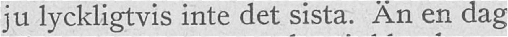ju lyckligtvis inte det sista. Än en dag
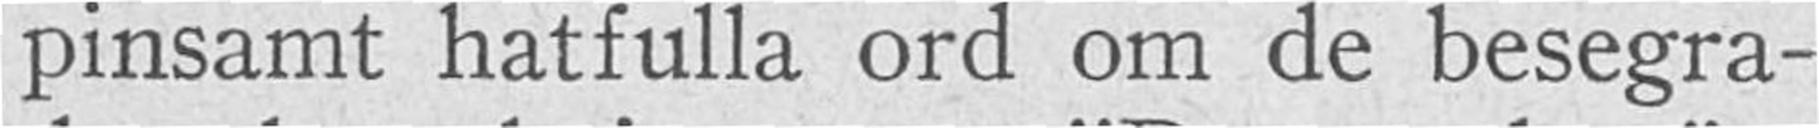pinsamt hatfulla ord om de besegra-
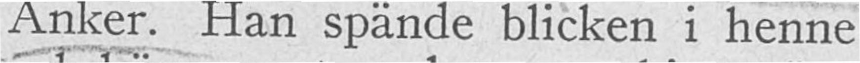Anker. Han spande blicken i henne
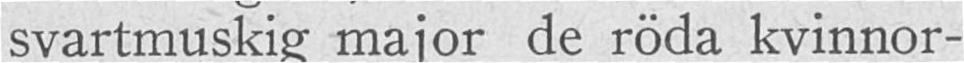svartmuskig major de röda kvinnor-
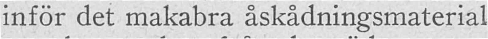inför det makabra åskådningsmaterial
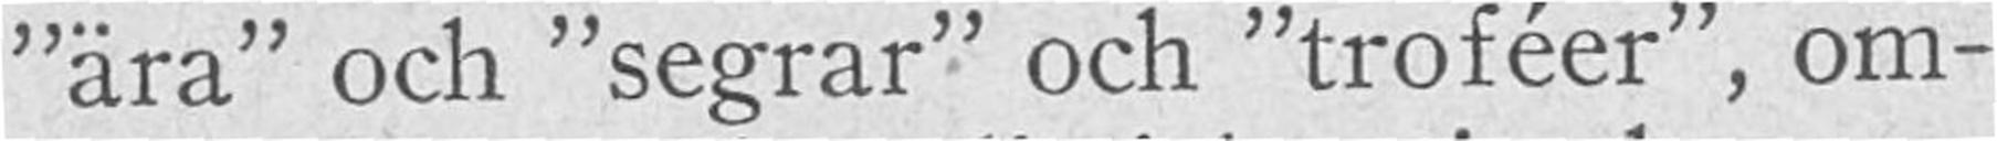"ära" och "segrar" och "troféer", om-
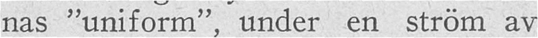nas "uniform" under en ström av
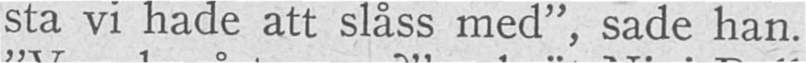sta vi hade att slåss med", sade han.
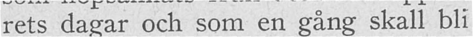rets dagar och som en gång skall bli
